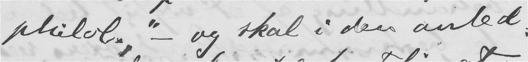philol.," - og skal i den anled-
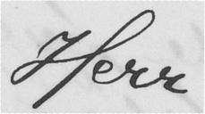Herr
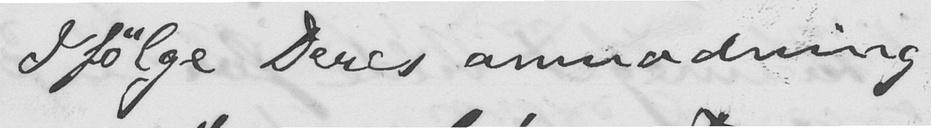Ifølge Deres anmodning
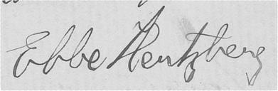Ebbe Hertzberg.
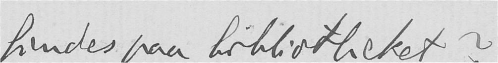findes paa bibliotheket?
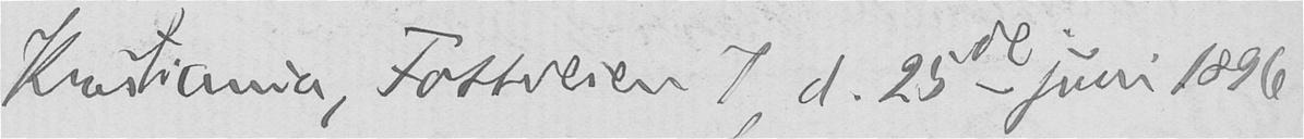Kritiania, Fossveien 7, d. 25de juni 1896.
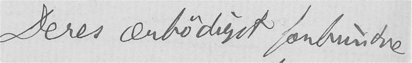Deres ærbødigst forbundne
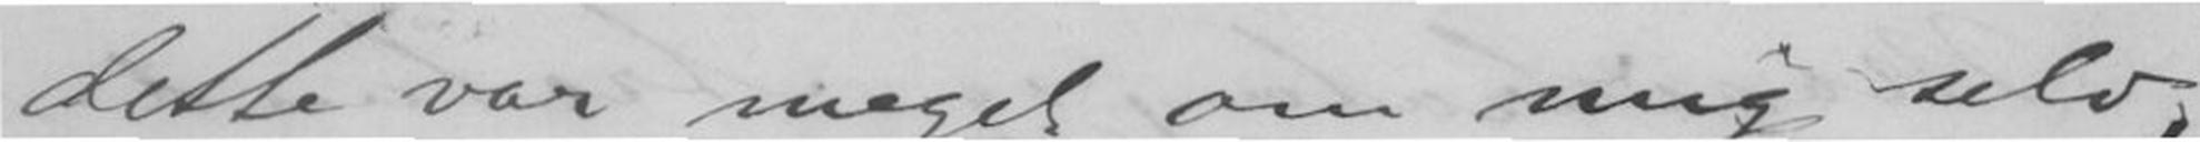dette var meget om mig selv,
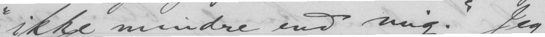"ikke mindre end mig". Jeg
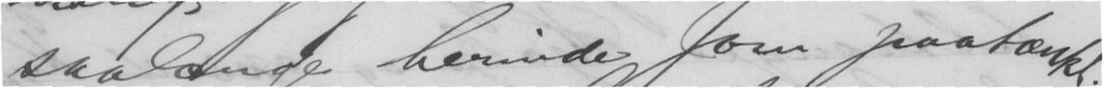saalænge herinde som paatænkt.
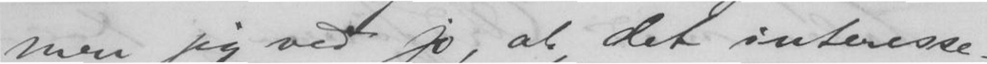men jeg ved jo, at det interesse-
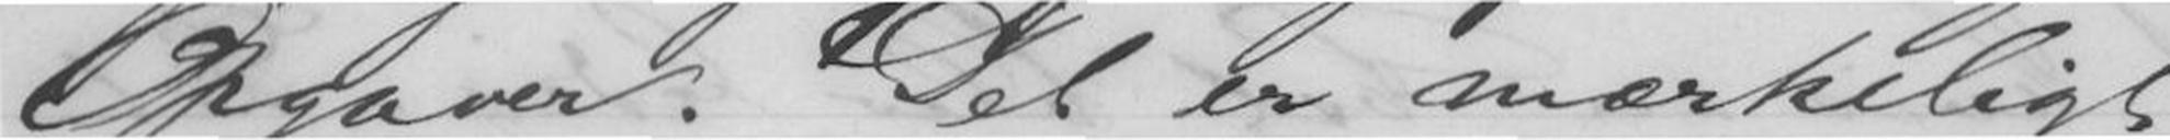Opgaver. Det er mærkeligt
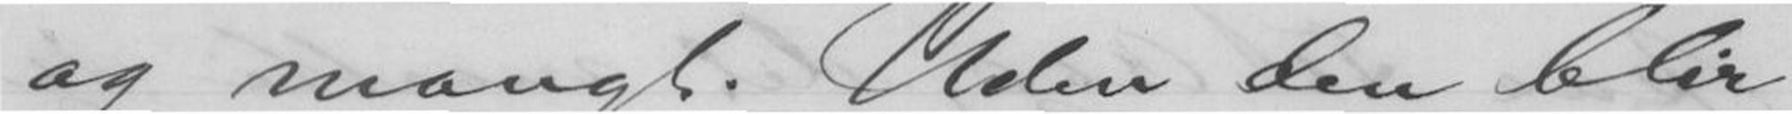og mangt. Uden den blir
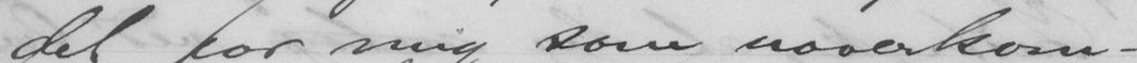det for mig som uoverkom-
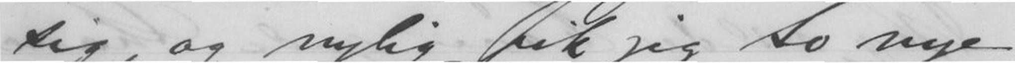sig, og nylig fik jeg to nye
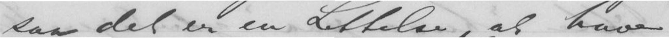saa det er en Lettelse, at have
